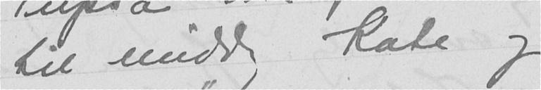til middag Kate og
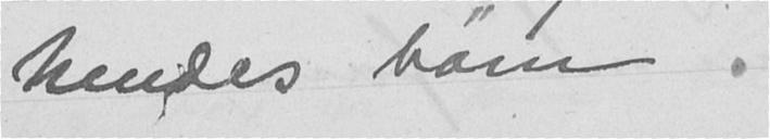hendes børn.
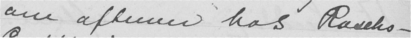om aftenen hos Raschs -
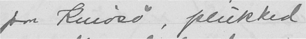paa Knivsø, plukked
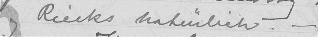og Riecks naturlich.
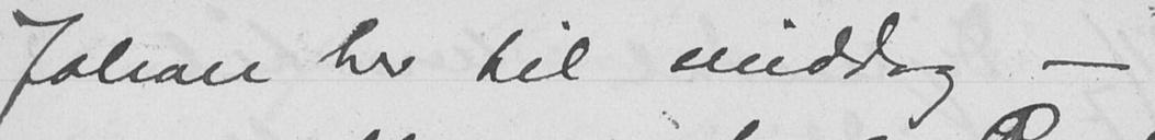Johan her til middag -
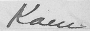Kom
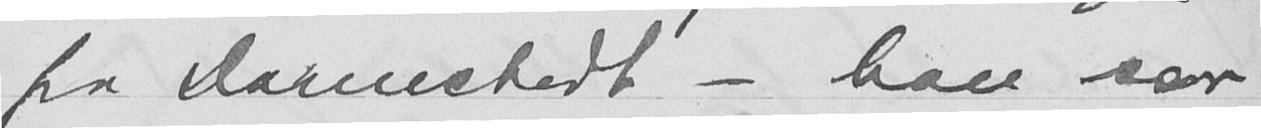fra Darmstadt - han saa
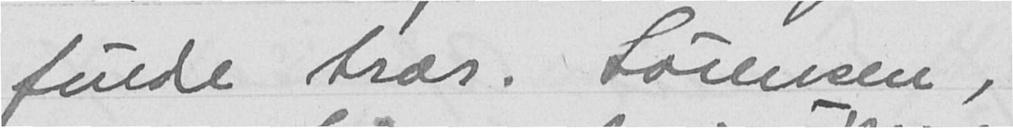fulde trær. Sørensen,
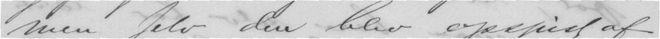men selv den blev opspist af
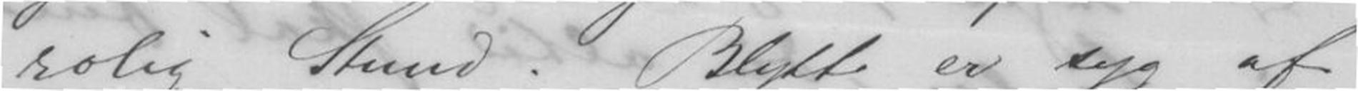rolig Stund. Blytt er syg af
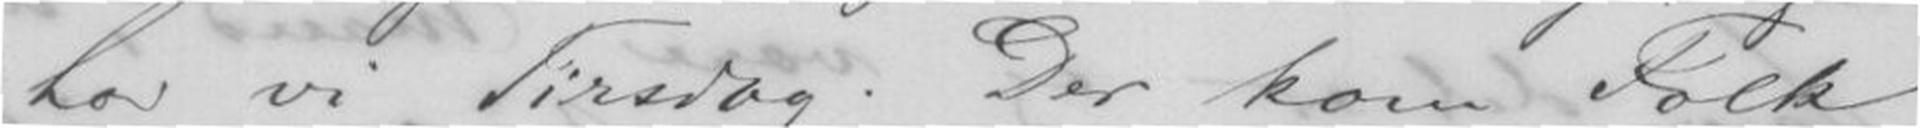har vi Tirsdag. Der kom Folk
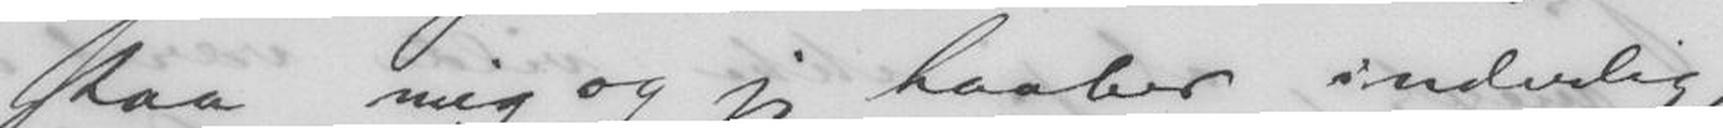staa mig og jeg haaber inderlig,
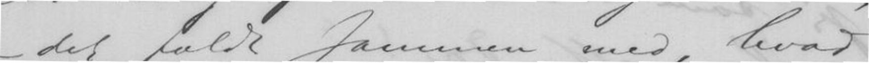- det faldt sammen med, hvad
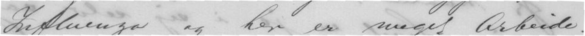Influenza og her er meget Arbeide.
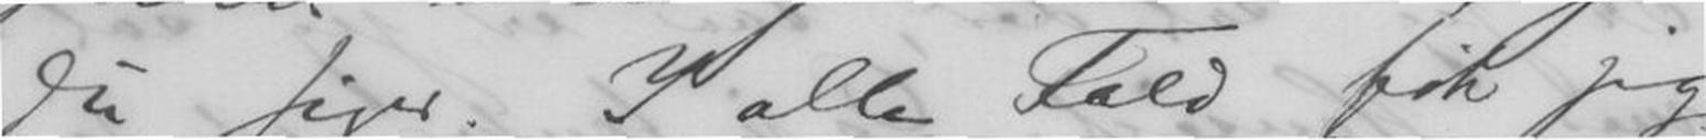du siger. I alle Fald fik jeg
